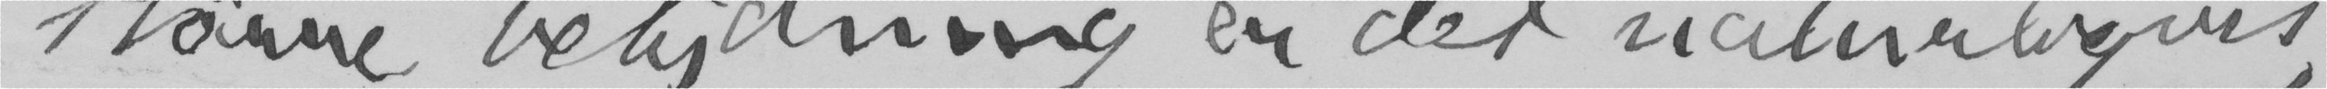større betydning er det naturligvis,
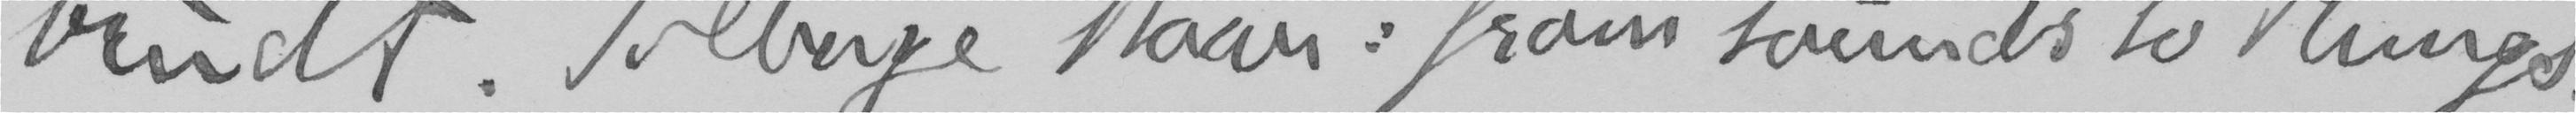brudt. Tilbage staar: from sounds to things.
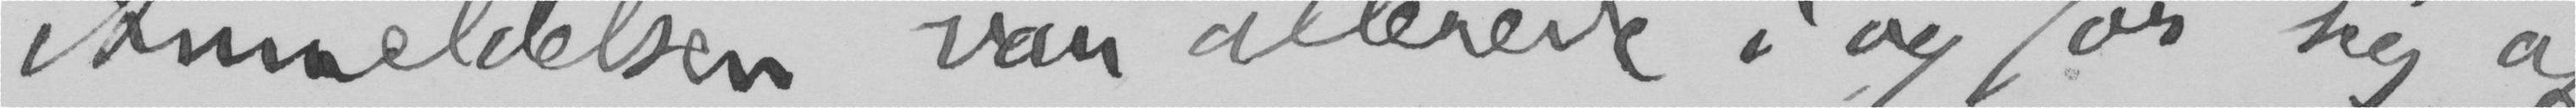Anmeldelsen var allerede i og for sig af
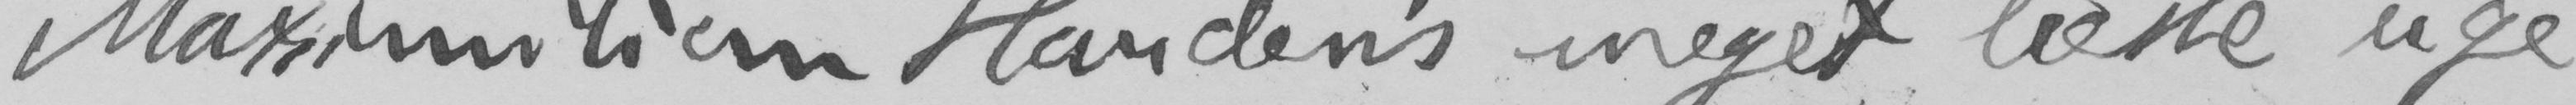Maximilian Harden’s meget læste uge-
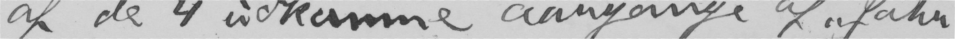af de 4 udkomne aargange af «Jahr-
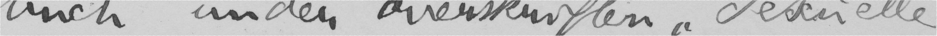buch» under overskiften «Sexuelle
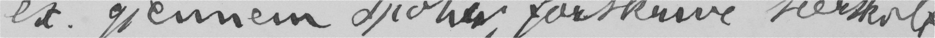ex. gjennem Spohr, forskrive særskilt.
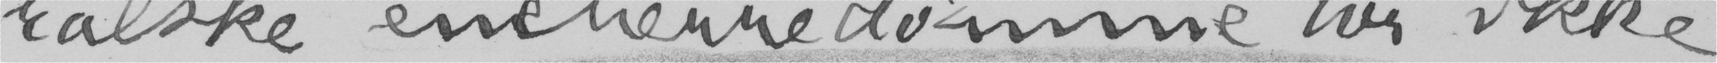ralske eneherredømme tør ikke
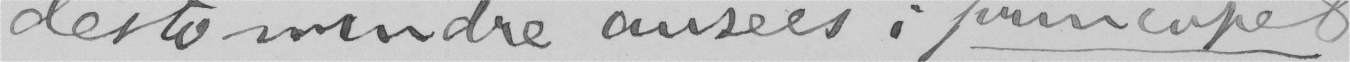desto mindre ansees i principet
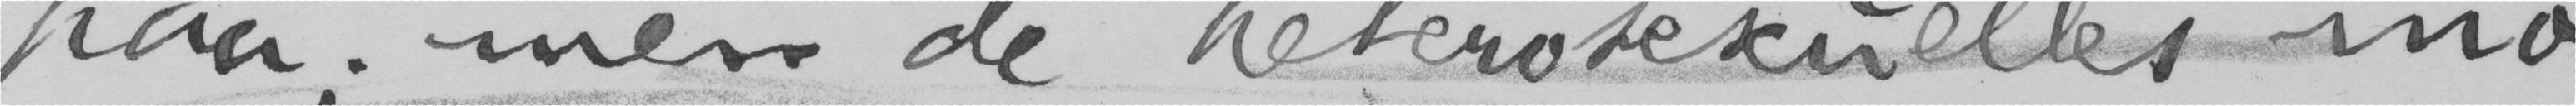paa; men de heterosexuelles mo-
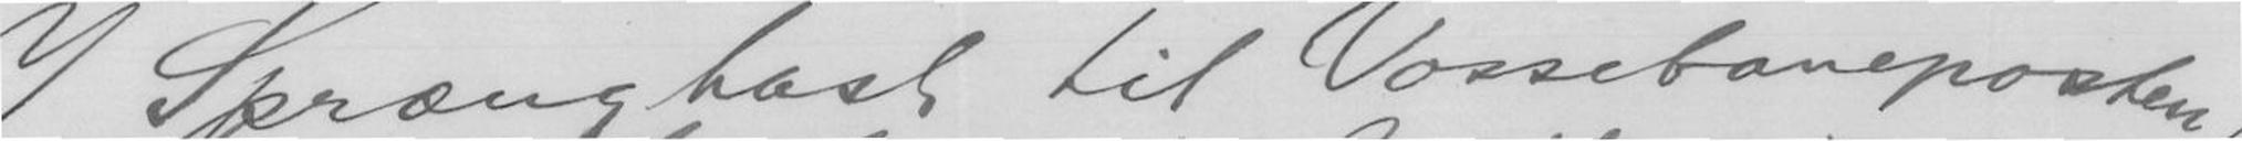I Spræng hast Vossebaneposten!
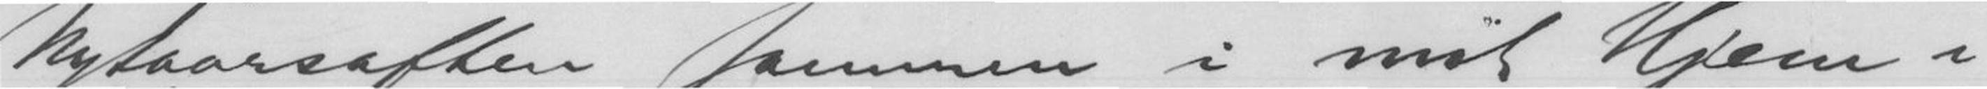Nytaarsaften sammen i mit Hjem i
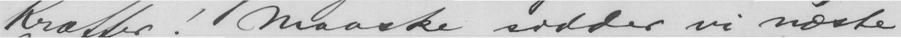Kræfter! Maaske sidder vi næste
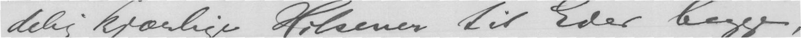delig kjærlige Hilsener til Eder begge,
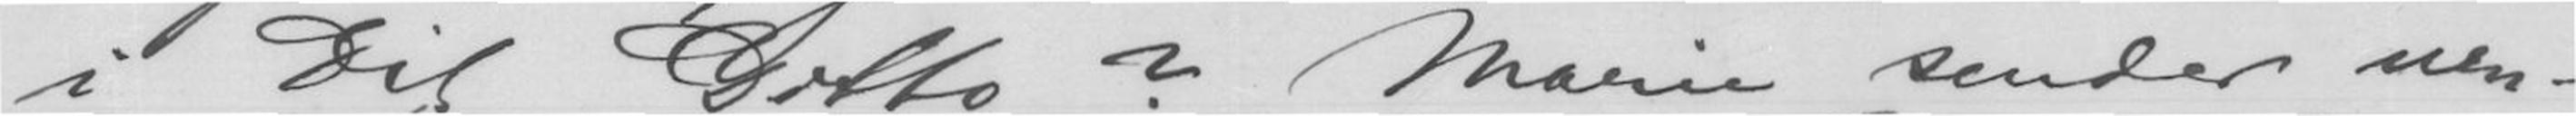i Dit Ditto? Marie sender uen-
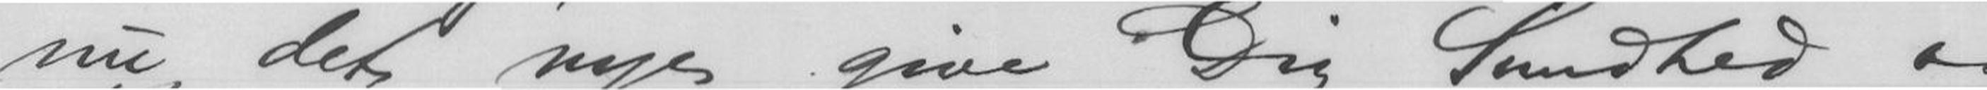nu det nye give Dig Sundhed og
